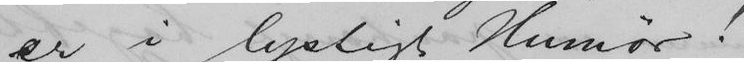er i lystigt Humør!
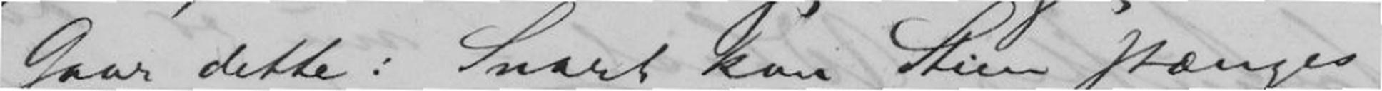Gaar dette: Snart kan Stien stænges
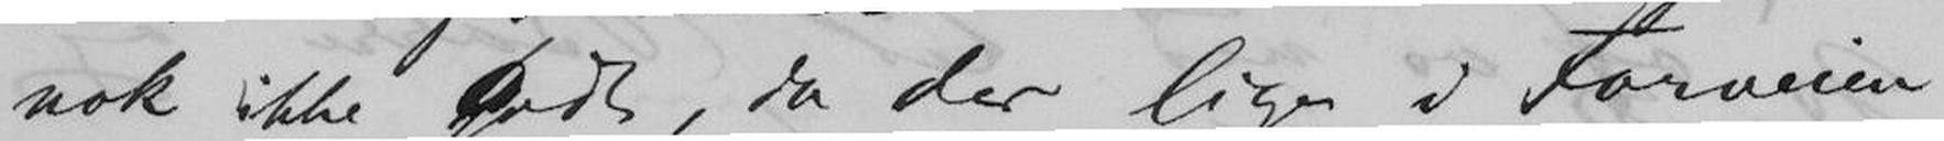nok ikke godt, da der lige i Forveien
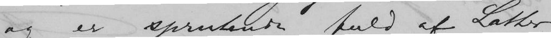og er sprutende fuld af Latter
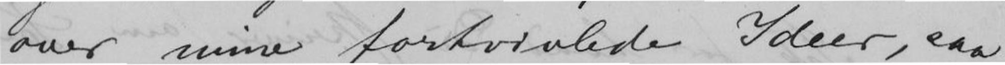over mine fortvivlede Ideer, saa
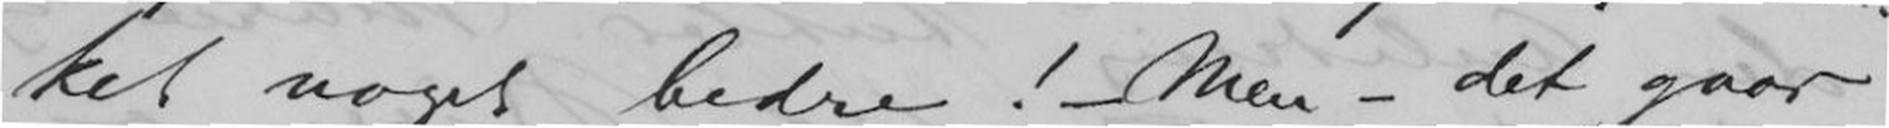ket noget bedre! - Men - det gaar
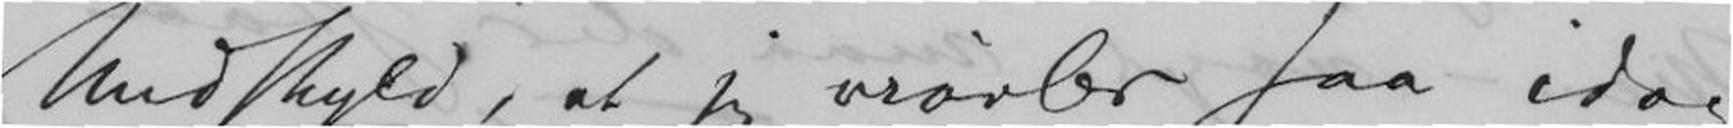Undskyld, at jeg vrøvler saa idag,
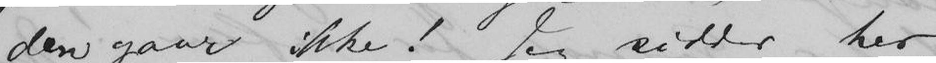den gaar ikke! Jeg sidder her
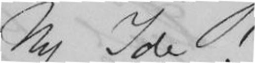Ny Ide!
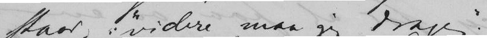staar, "videre maa jeg drages."
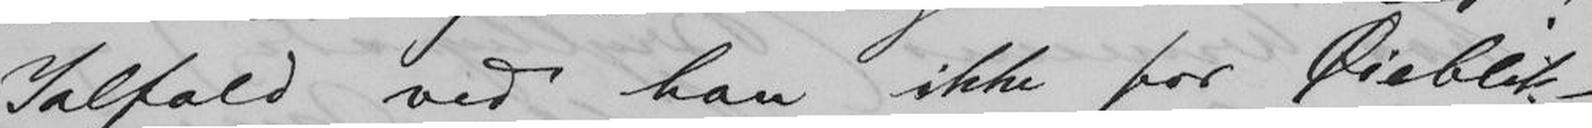Ialfald ved han ikke for Øieblik-
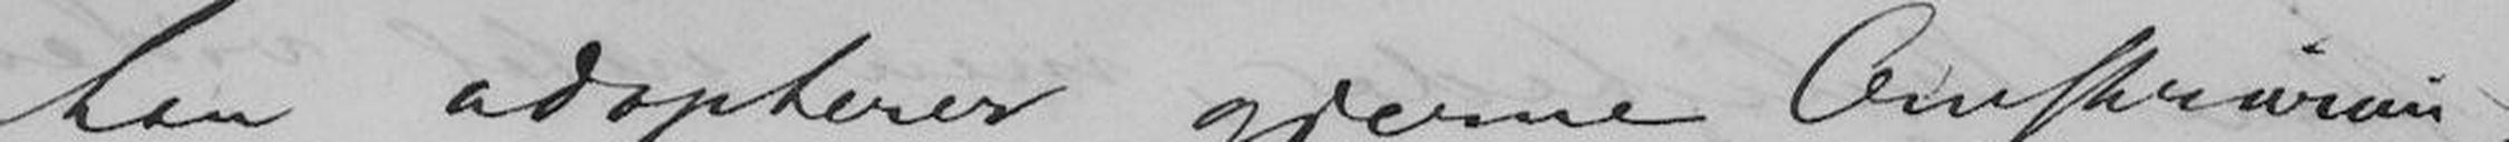han adopterer gjerne Omskrivnin-
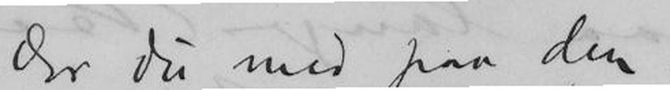Er Du med paa den!
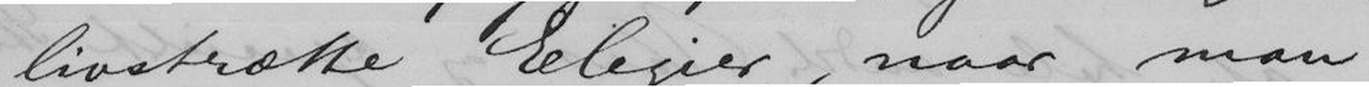livstrætte Elegier, naar man
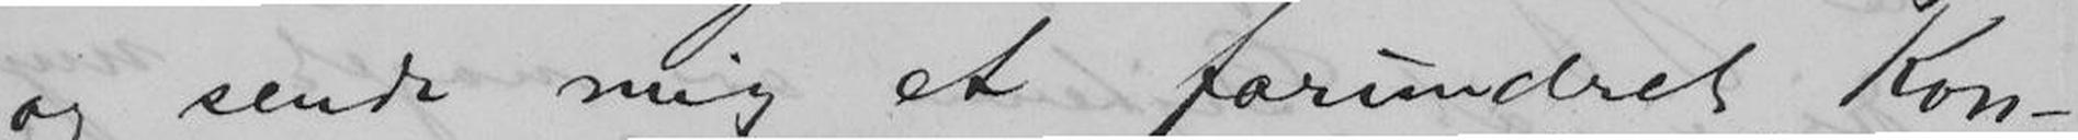og sende mig et forundret Kon-
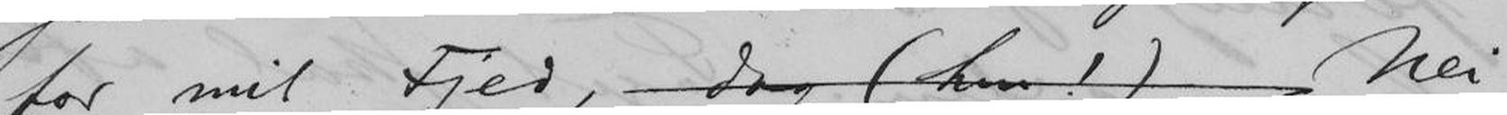for mit Fjed, dog (hm!) Nei
